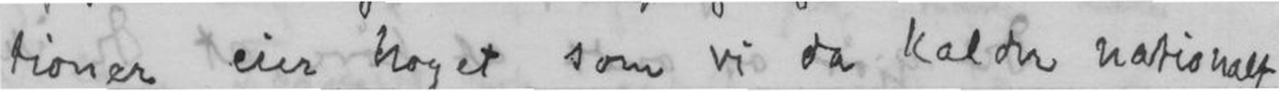tioner eier noget som vi da kalder nationalt,
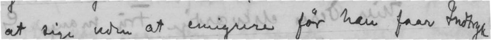at sige uden at emigrere før han faar Indtrykk.
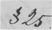§ 25
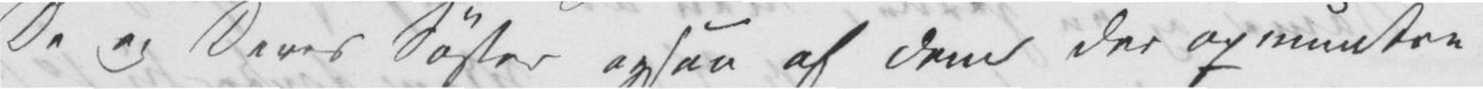De og Deres Søster ogsaa af dem der opmuntre
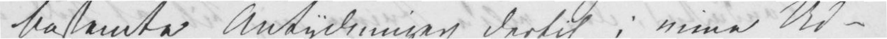bestemte Antydninger dertil i mine Ud¬
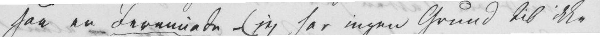saa en Jeremiade – (jeg har ingen Grund til ikke
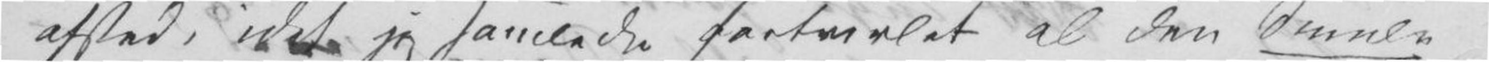afsted, idet jeg samlede fortvivlet al den Smule
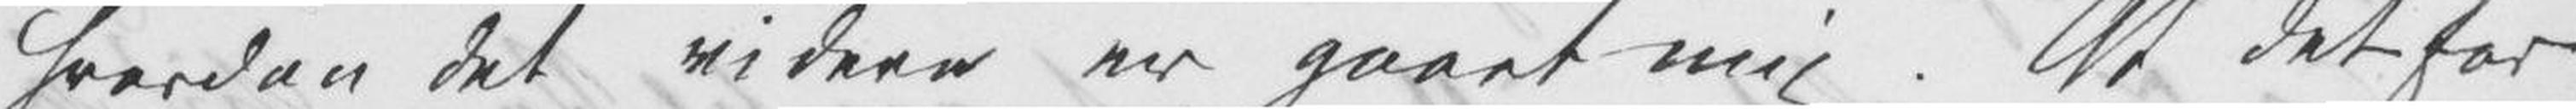hvordan det videre er gaaet mig. At det for
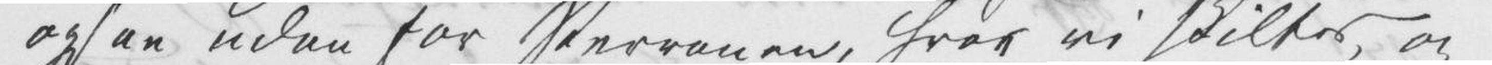ogsaa uden for Perronen, hvor vi skiltes, og
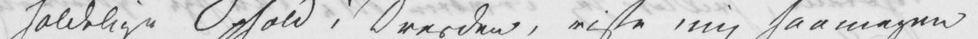holdelige Ophold i Dresden, viste mig saamegen
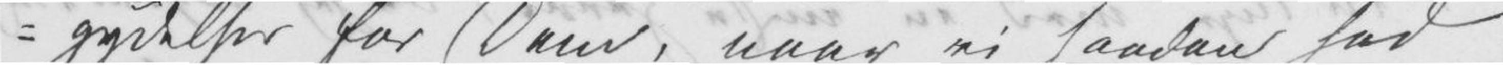gydelser for Dem, naar vi saadan sad
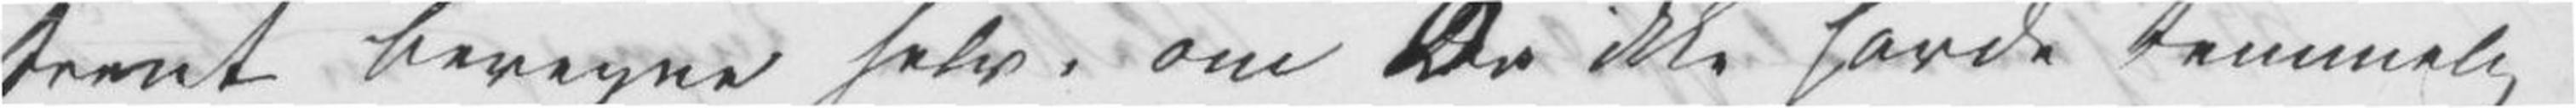trent beregne selv, om De ikke havde temmelig
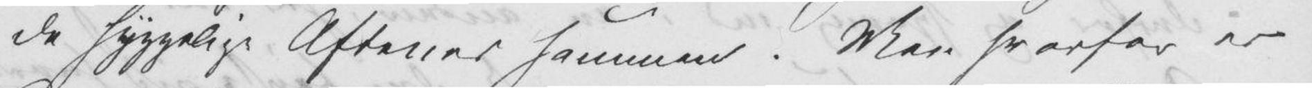de hyggelige Aftener sammen. Men hvorfor er
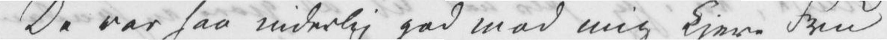De var saa inderlig god mod mig kjere Fru
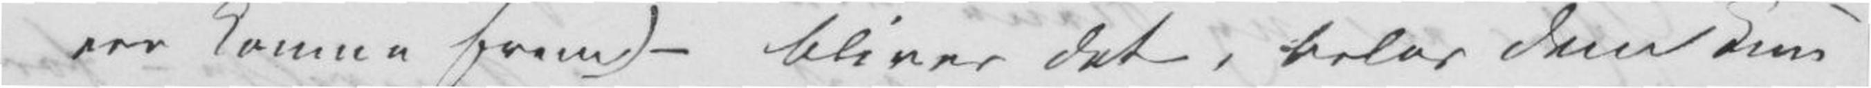ere komne frem) – bliver det, belav Dem kun
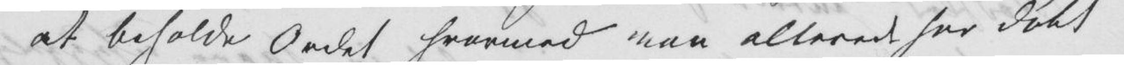at beholde Ordet hvormed man allerede har døbt
